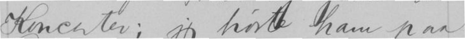Koncerter; jeg hørte ham paa
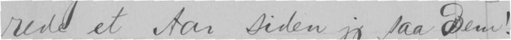rede et Aar siden jeg saa Dem!
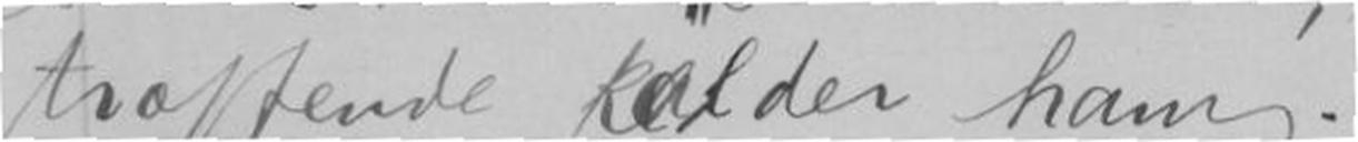træffende kalder ham.
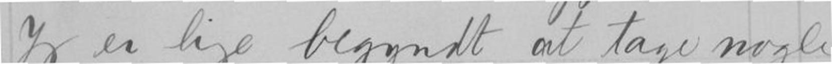Jeg er lige begyndt at tage nogle
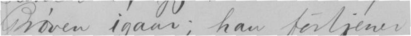Prøven igaar; han fortjener
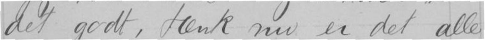det godt, tænk nu er det alle-
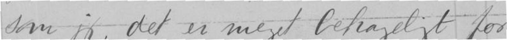som jeg, det er meget behageligt for
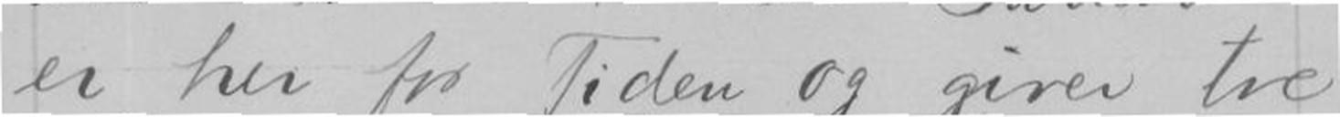er her for Tiden og giver tre
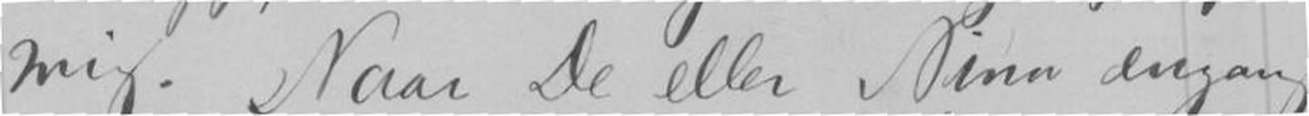mig. Naar de eller Nina engang
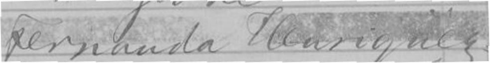Fernanda Henriques
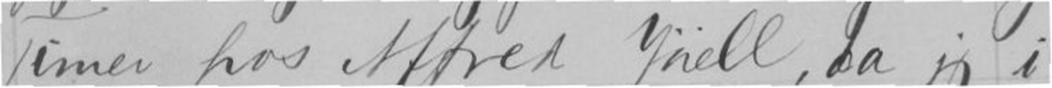Timer hos Alfred Jüell, da jeg i
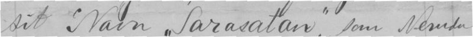sit Navn Sarasatan, som Neruda
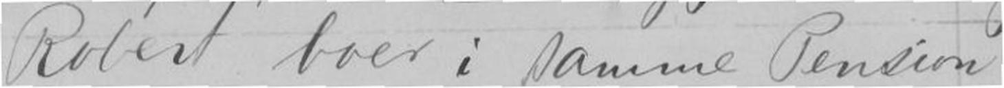Robert boer i samme Pension
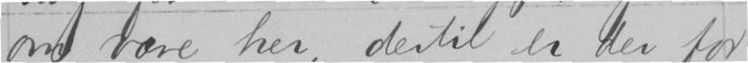om være her, dertil er der for
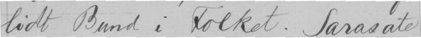lidt Bund i Folket. Sarasate
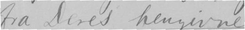fra Deres hengivne
